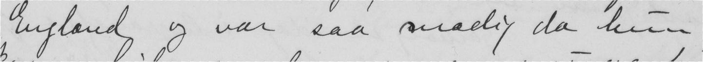England og var saa modig da hun
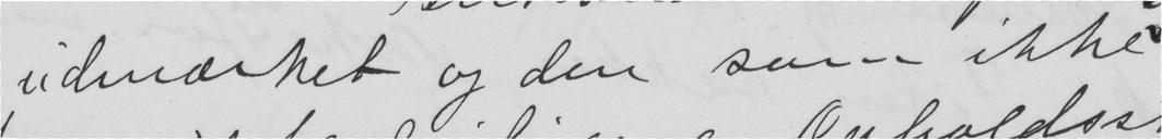udmærket og den som ikke
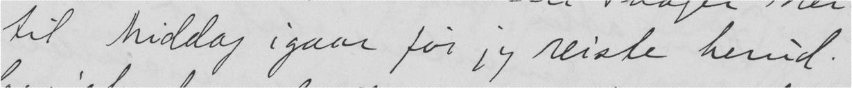til Middag igaar før jeg reiste herud.
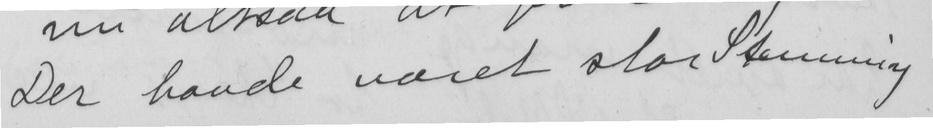Der havde været stor Stemning
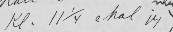Kl. 11 1/4 skal jeg
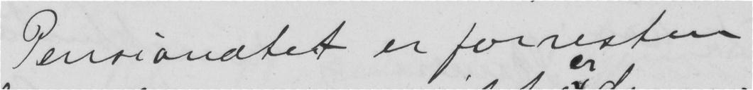Pensionatet er forresten
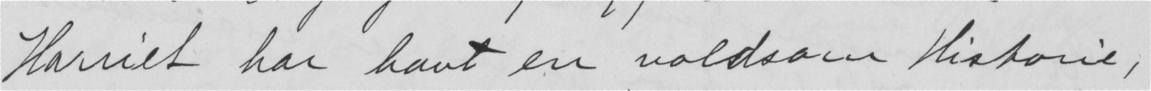Harriet har havt en voldsom Historie,
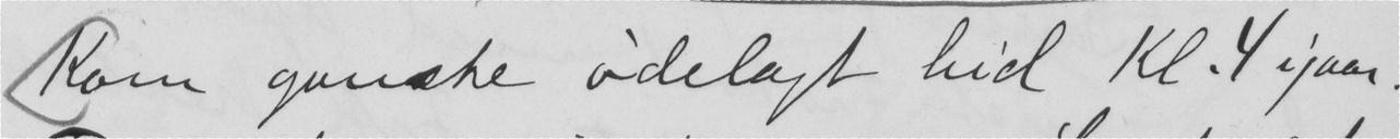Kom ganske ødelagt hid Kl. 4 igaar.
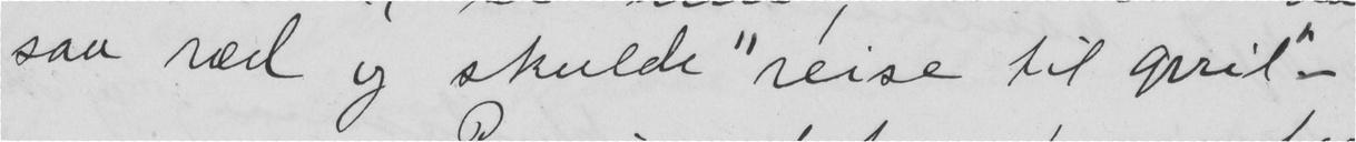saa ræd og skulde "reise til april" -
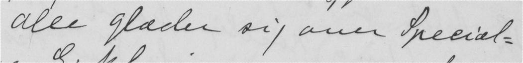Alle glæder sig over Special-
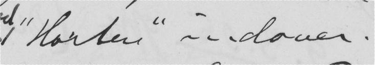Horten indover.
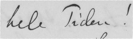hele Tiden!
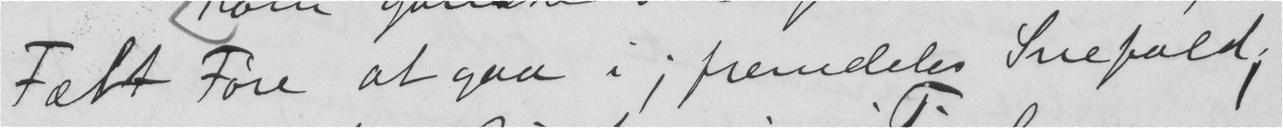Fælt Føre at gaa i; fremdeles Snefald,
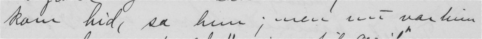kom hid, sa hun; men nu var hun
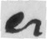er
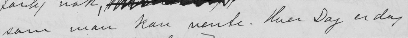som man kan vente. Hver Dag er dog
